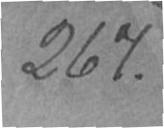267.
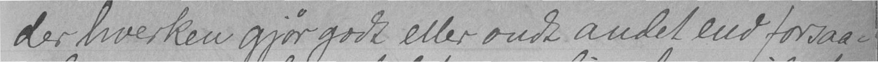der hverken gjør godt eller ondt andet end forsaa-
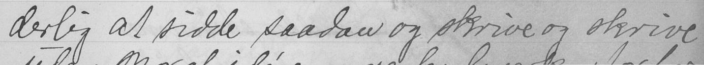derlig at sidde saadan og skrive og skrive
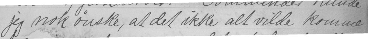jeg nok ønske, at det ikke alt vilde komme
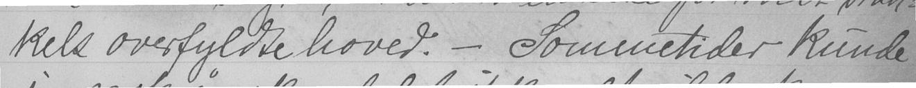kels overfyldte hoved. - Sommetider kunde
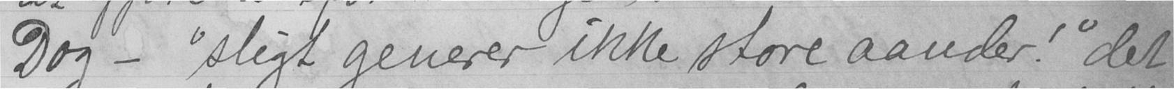Dog - "sligt generer ikke store aander!" det
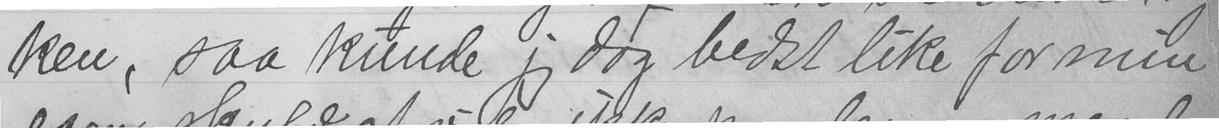ken, saa kunde jeg dog bedst like for min
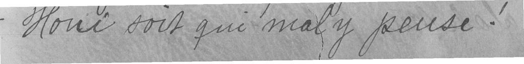- "Honi soit qui mal y pense!
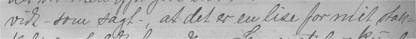vidt - som sagt -, at det er en lise for mit stak-
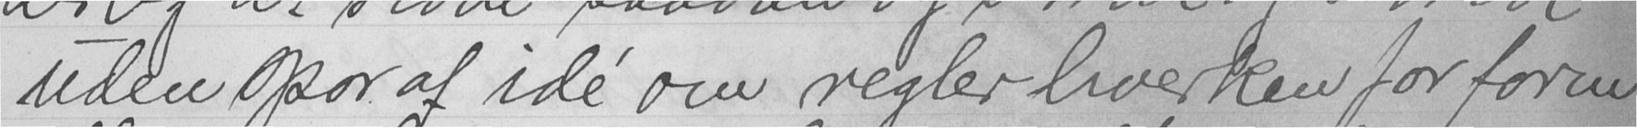uden spor af idé om regler hverken for form
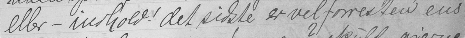eller - indhold! det sidste er vel forresten ens
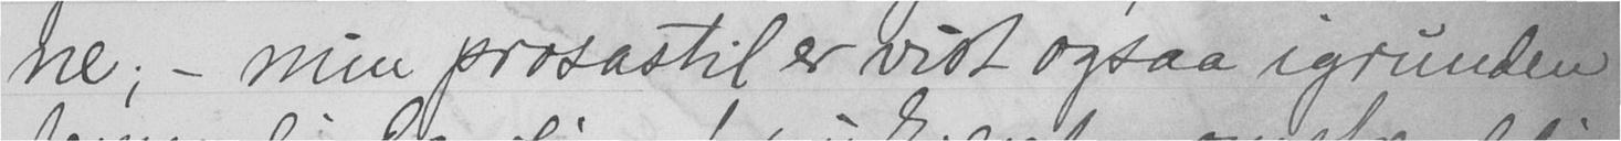ne; - min prosastil er vist ogsaa igrunden
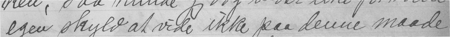egen skyld at vide ikke paa denne maade
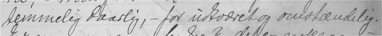temmelig daarlig, - for udtværet og omstændelig.
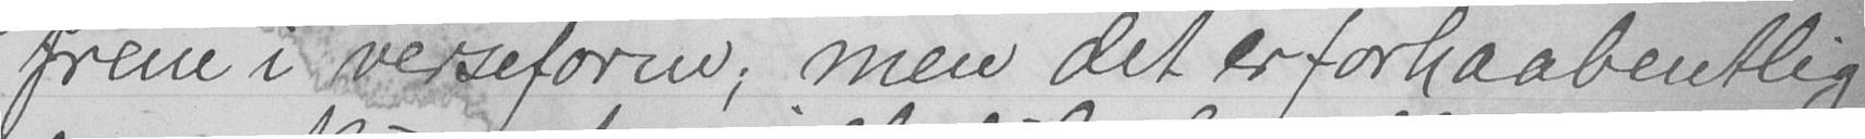frem i verseform; men det er forhaabentlig
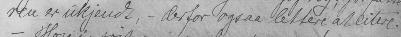ren er ukjendt, - derfor ogsaa lettere at citere.
